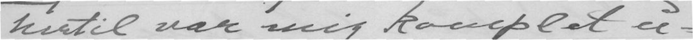hertil var mig komplet u-
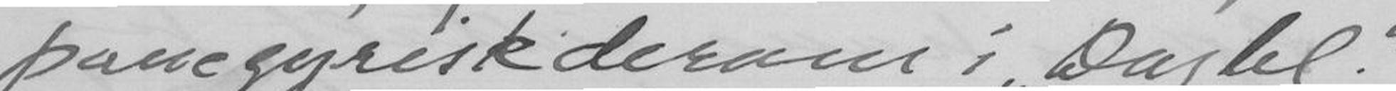panegyrisk derom i "Dagbl"
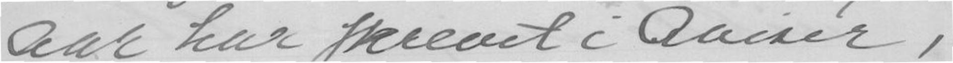Aar har skrevet i Aviser,
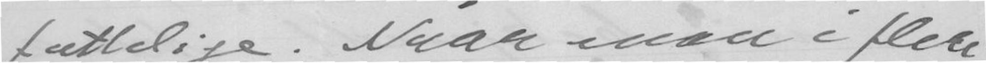fattelige. Naar man i flere
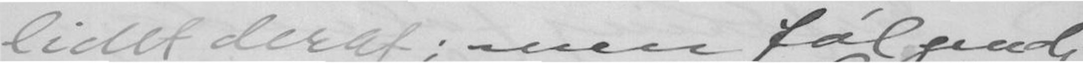lidet deraf; men følgende
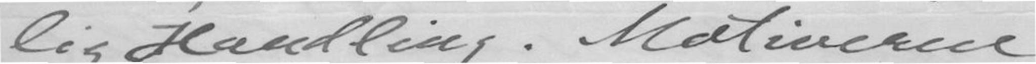lig Handling. Motiverne
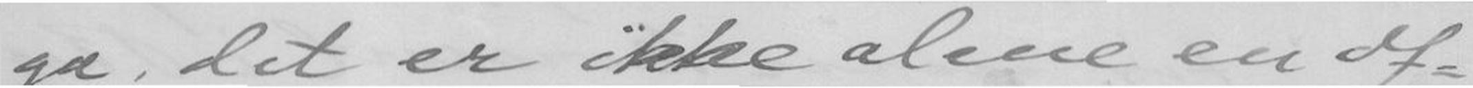ga, det er ikke alene en of-
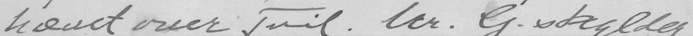hævet over Tvil. Hr. G. skylder
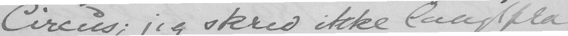Circus; jeg skrev ikke langt fra
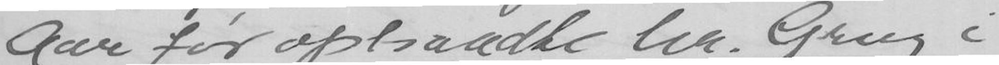Aar før optraadte hr. Grieg i
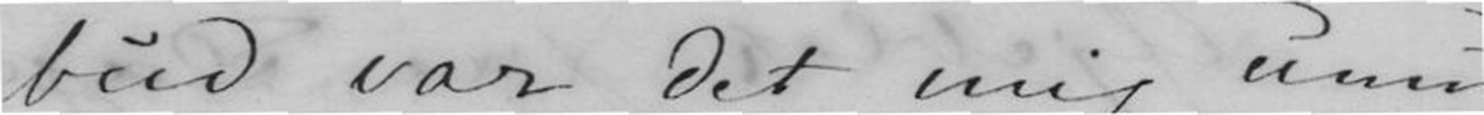bud var det mig umu-
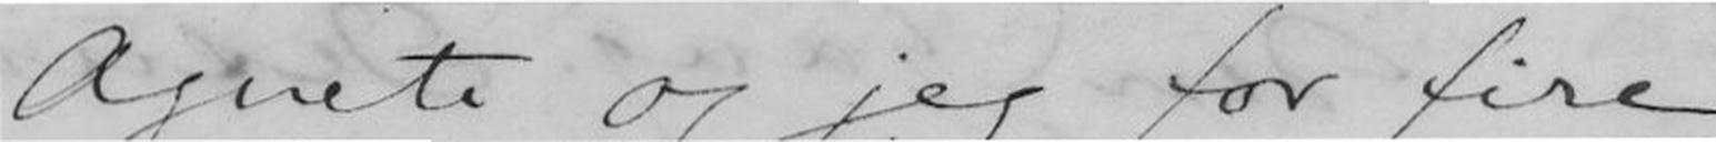Agnete og jeg for fire
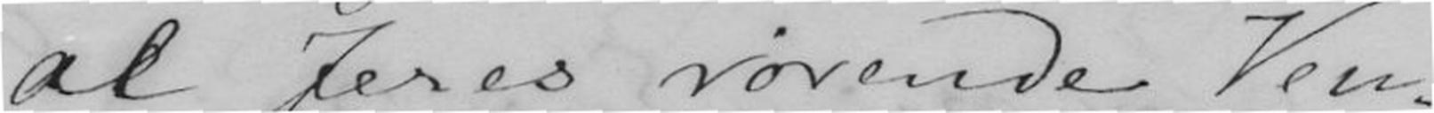al Jeres rørende Ven-
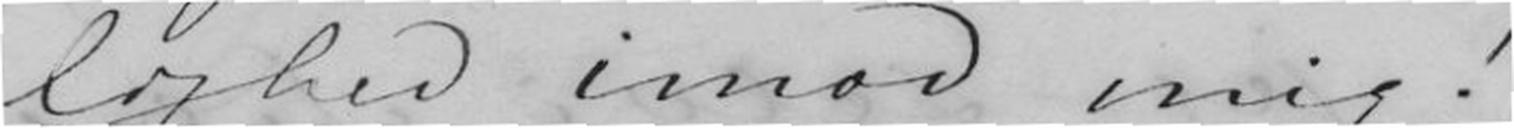lighed imod mig!
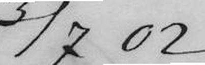3/7 02
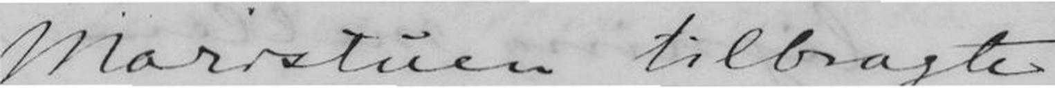Maristuen tilbragte
